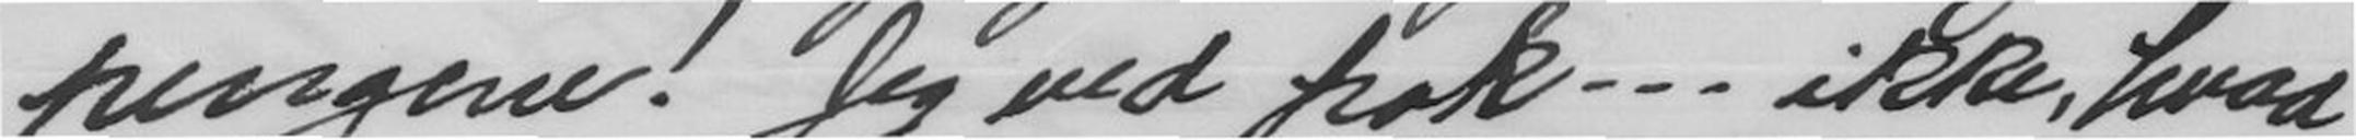pengene! Jeg ved pok--- ikke, hvad
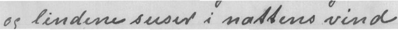og lindene suser i nattens vind,
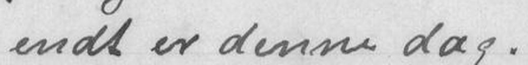endt er denne dag.
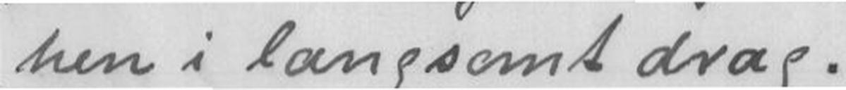hen i langsomt drag.
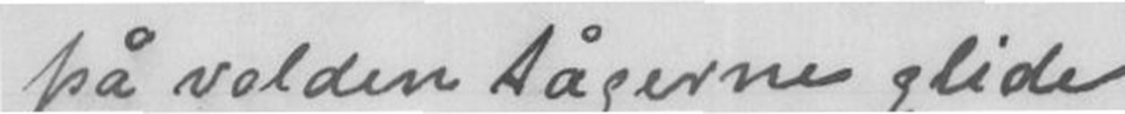på volden tågerne glide
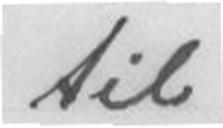til
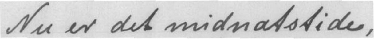Nu er det midnatstide,
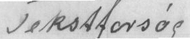Tekstforsøg
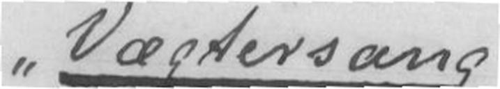Vægtersang
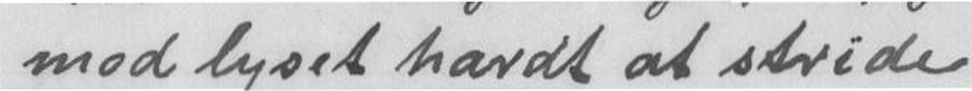Mod lyset hardt at stride
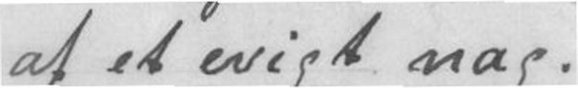af et evigt nag.
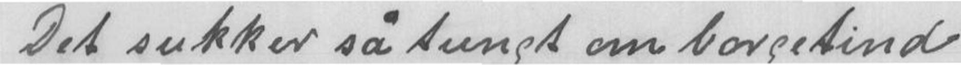Det sukker så tungt om borgetind
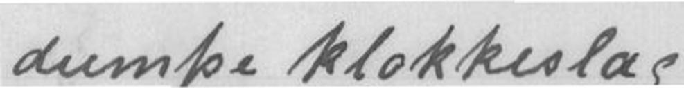dumpe klokkeslag
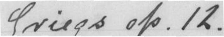Griegs op. 12.
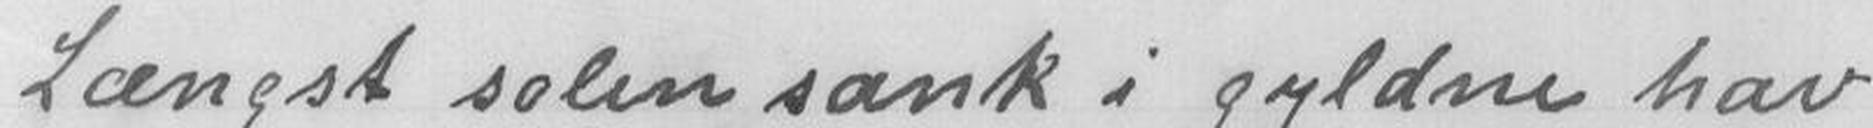Langst solen sank i gyldne hav
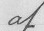af
